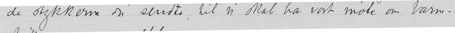de stykkerne du sendte, til vi skal ha vort møte om barne-
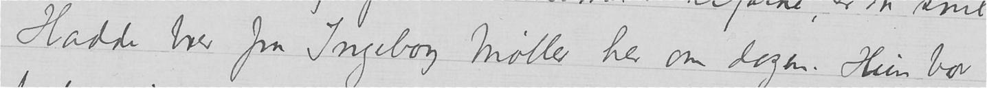Hadde brev fra Ingeborg Møller her om dagen. Hun bor
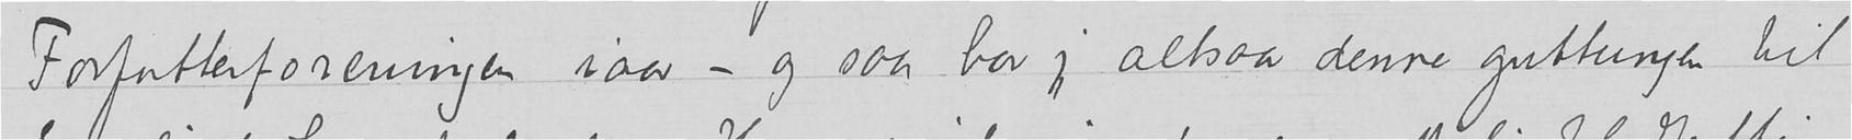Forfatterforeningen iaar – og saa har jeg altsaa denne guttungen til
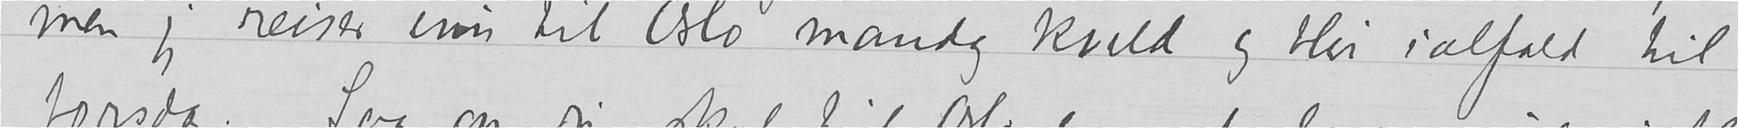men jeg reiser nu til Oslo mandag kveld og blir ialfald til
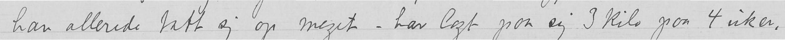han allerede tatt sig op meget – har lagt paa sig 3 kilo paa 4 uker,
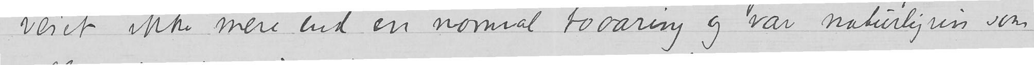veiet ikke mere end en normal toaaring og var naturligvis som
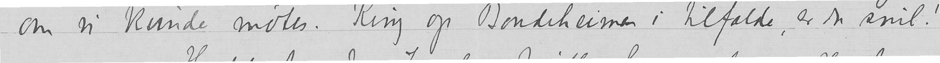om vi kunde møtes. Ring op Bondeheimen i tilfælde, er du snil!
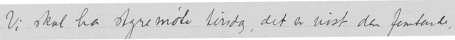Vi skal ha styremøte tirsdag, det er vist den femtende,
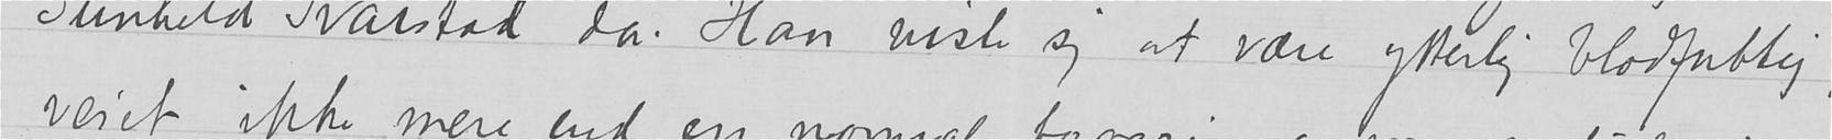Gunhild Svarstad da. Han viste sig at være ytterlig blodfattig,
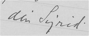din Sigrid
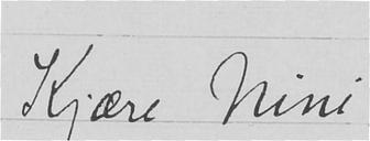Kjære Nini,
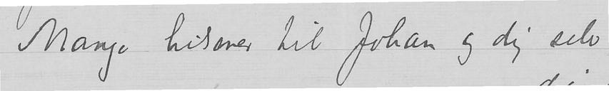Mange hilsener til Johan og dig selv
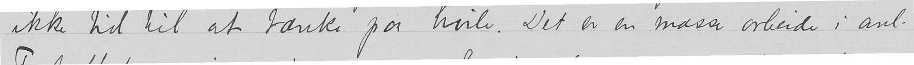ikke tid til at tænke paa hvile. Det er en masse arbeide i anl.
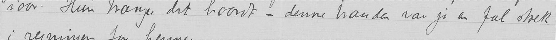iaar. Hun trænger det haardt – denne branden var jo en fæl strek
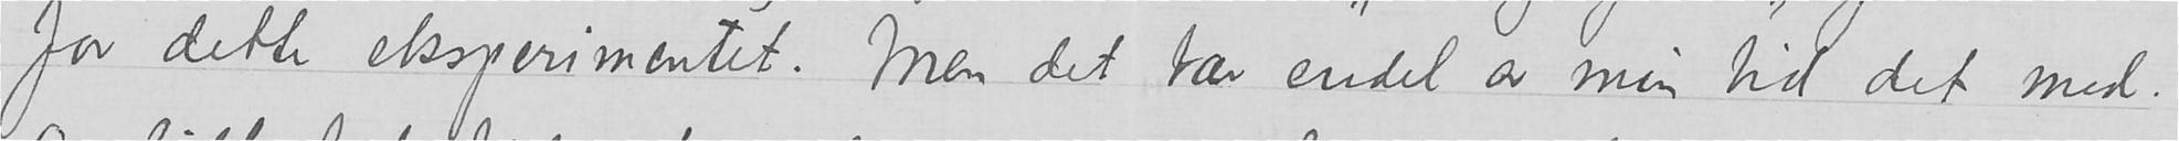for dette eksperimentet. Men det tar en del av min tid det med.
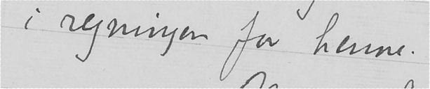i regningen for henne.
